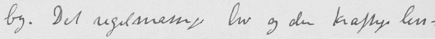by. Det regelmæssige liv og den kraftige leve-
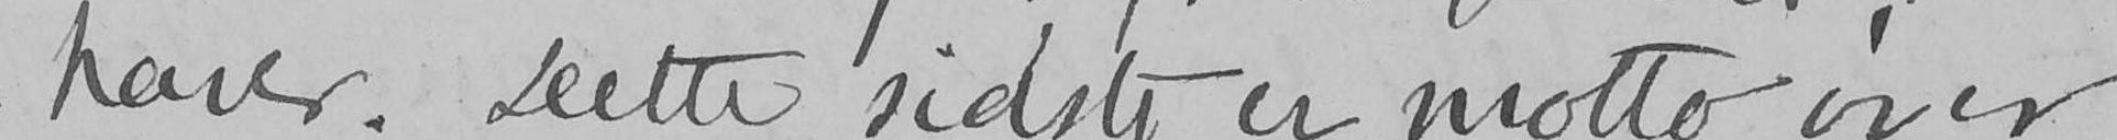haver. Dette sidste er motto over
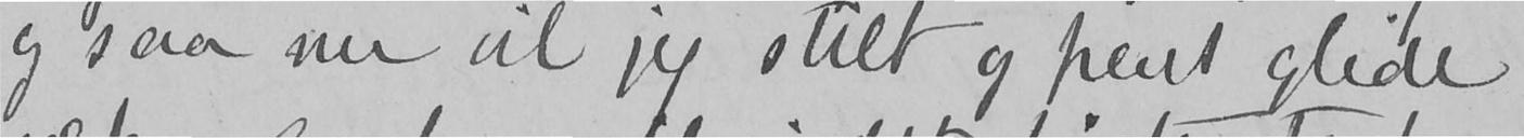og saa nu vil jeg stilt og pent glide
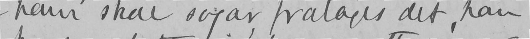ham skal sogar fratages det, han
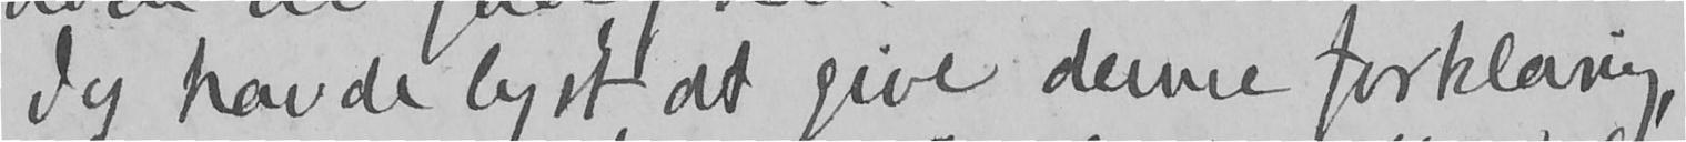Jeg havde lyst at give denne forklaring,
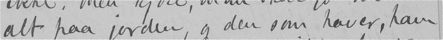alt paa jorden, og den som haver, ham
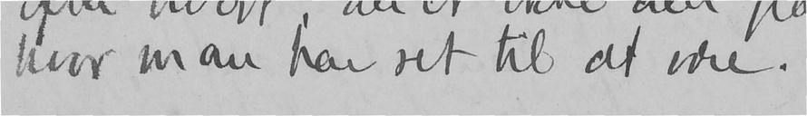hvor man har ret til at være.
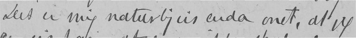Det er mig naturligvis enda ondt, at jeg
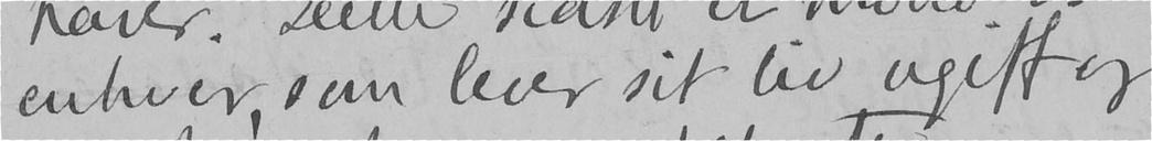enhver, som lever sit liv ugift og
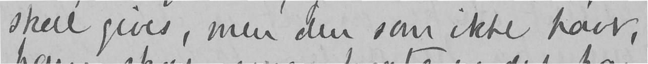skal gives, men den som ikke haver,
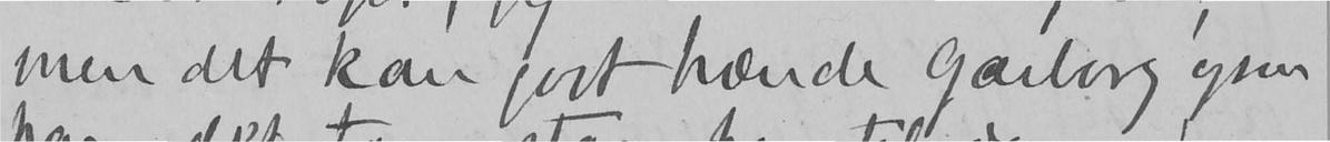men det kan godt hænde Garborg ogsaa
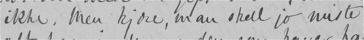ikke. Men kjære, man skal jo miste
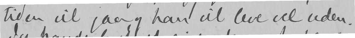tiden vil gaa, og han vil leve vel uden.
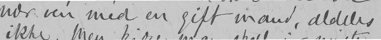nær ven med en gift mand, aldeles
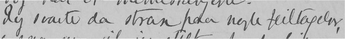Jeg svarte da strax paa nogle feiltagelser,
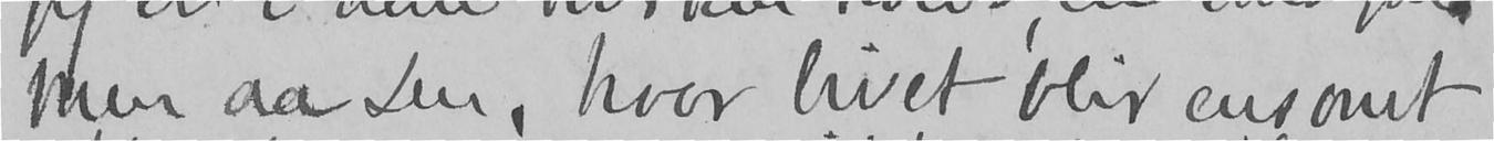Men aa Du, hvor livet blir ensomt
